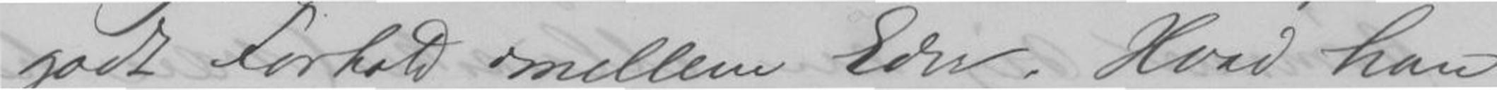godt Forhold mellem Eder. Hvad han
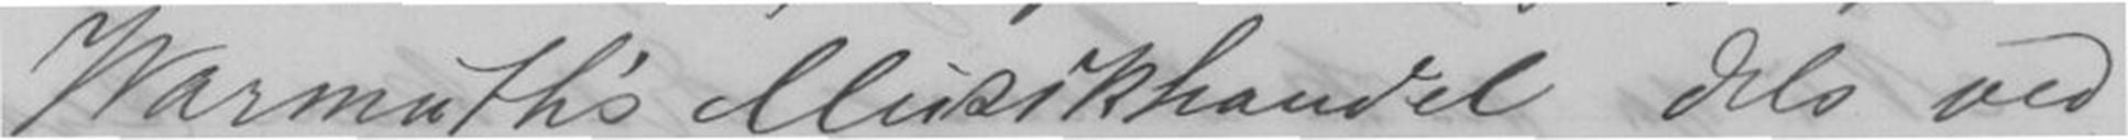Warmuth Musikhandel dels ved
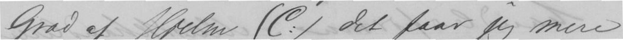Grad af Hjelm (C:) det faar jeg mere
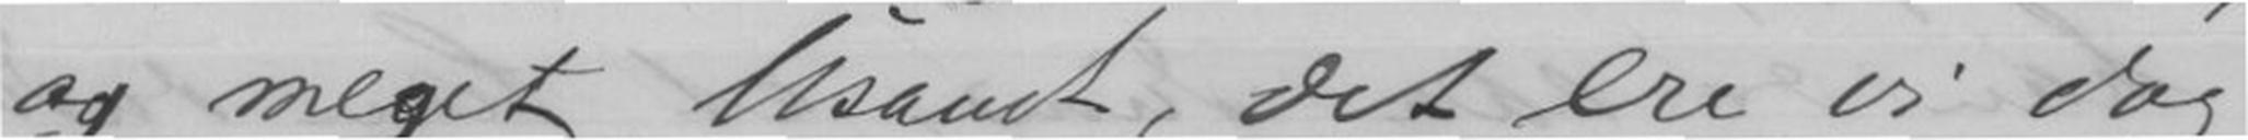og meget Usant, det ere vi dog
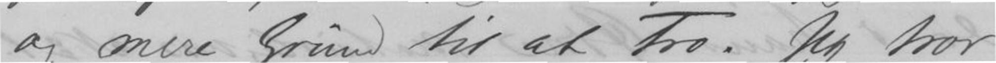og mere Grund til at tro. Jeg tror
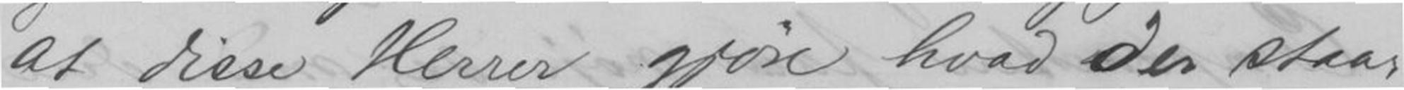at disse Herrer gjør hvad det staar
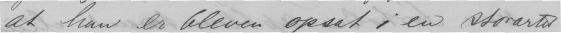at han er bleven opsat i en storartet
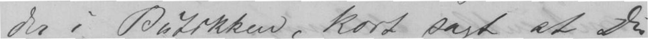der i Butikken, kort sagt at Du
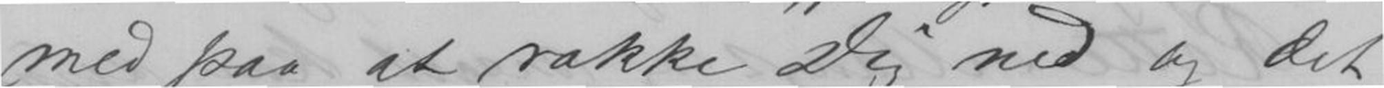med paa at rakke Dig ned og det
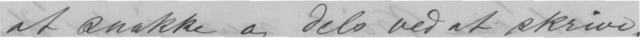at snakke og dels ved at skrive,
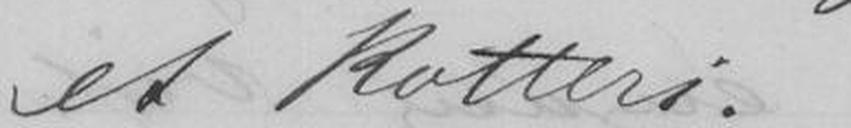et Kotteri.
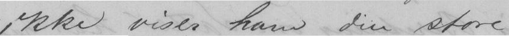ikke viser ham din store
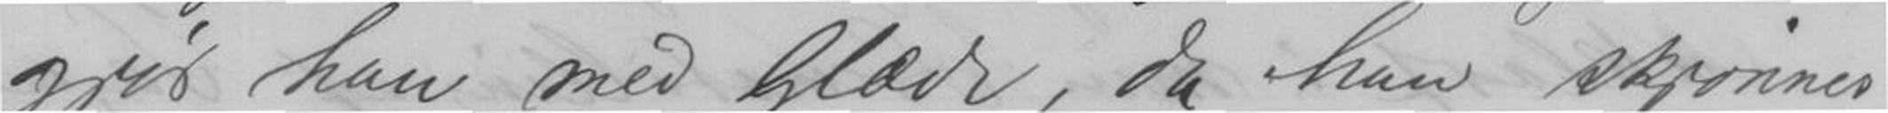gjør han med Glæde, da han skjønner
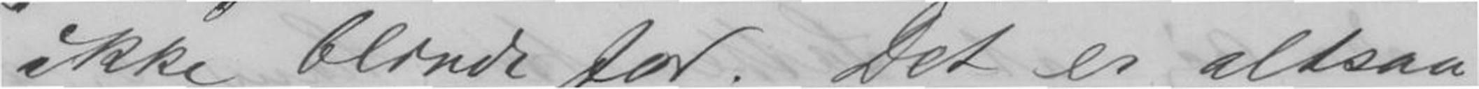ikke blinde for. Det er altsaa
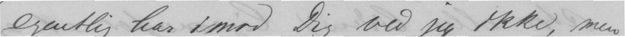egentlig har imod Dig ved jeg ikke, men
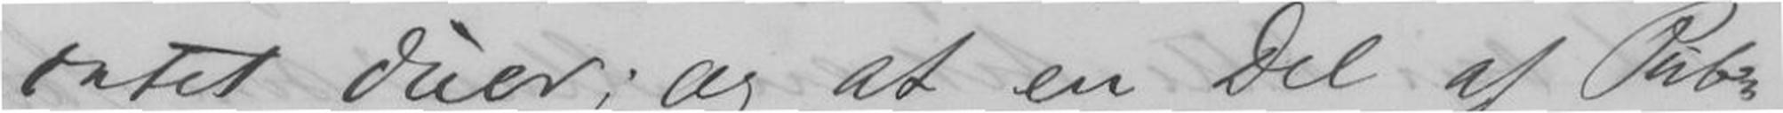intet Duer; og at en Del af Publ-
